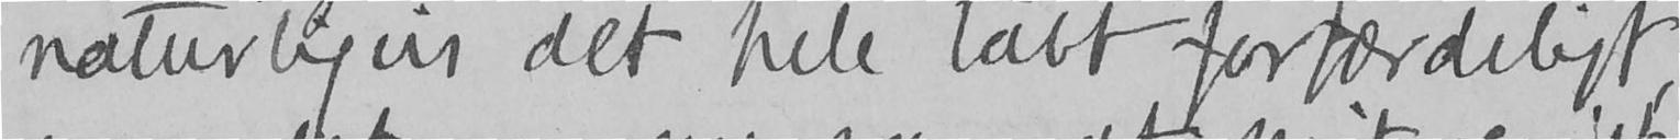naturligvis det hele tabt forfærdeligt,
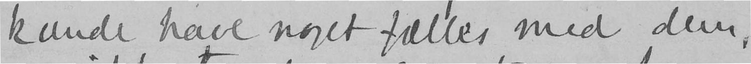kunde have noget fælles med dem,
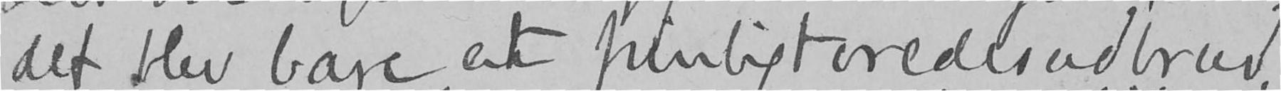det blev bare et pinligt vredesudbrud,
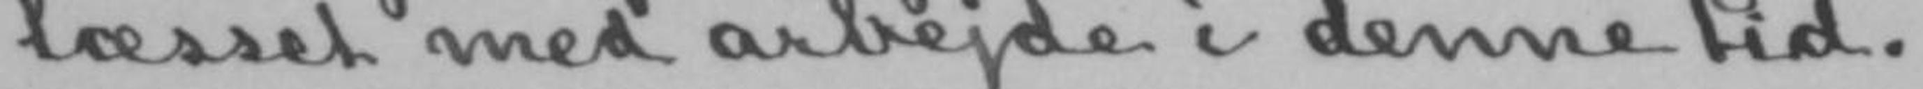læsset med arbejde i denne tid.
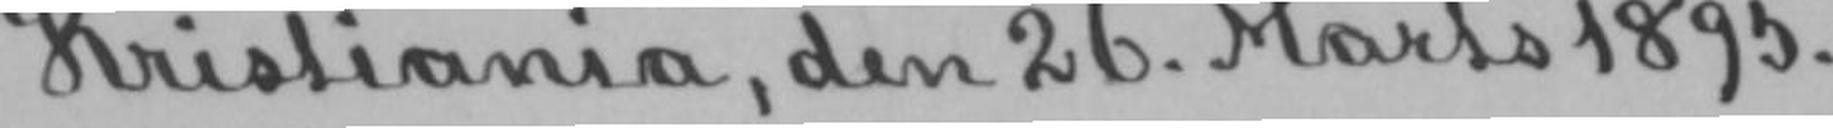Kristiania, den 26. Marts 1895.
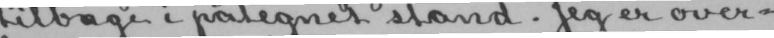tilbage i påtegnet stand. Jeg er over-
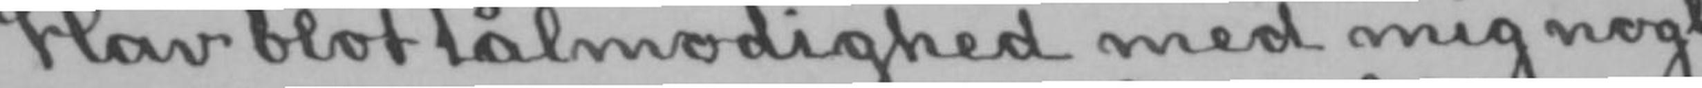Hav blot tålmodighed med mig nogle
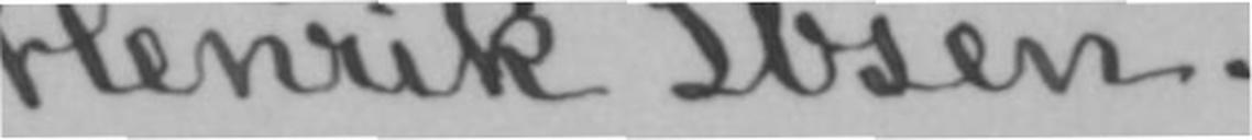Henrik Ibsen.
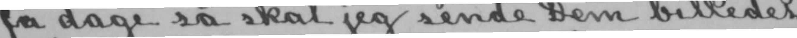få dage så skal jeg sende Dem billedet
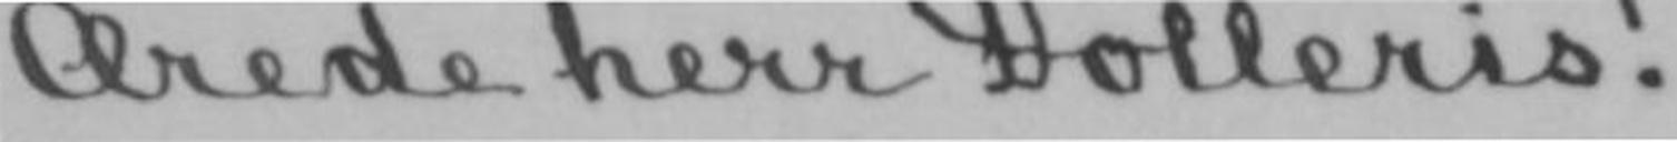Ærede herr Dolleris!
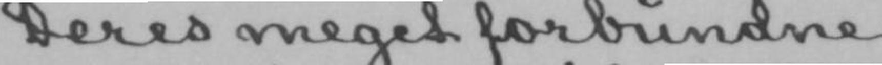Deres meget forbundne
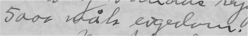5000 måls eigedom.
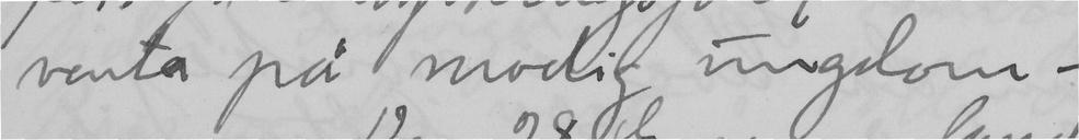vente på modig ungdom –
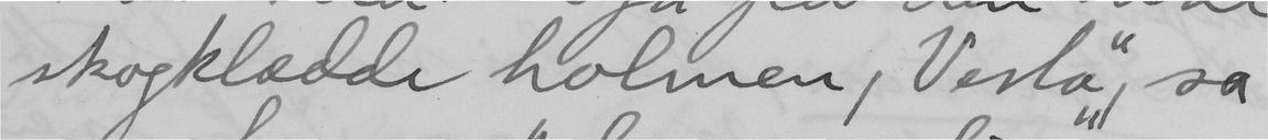skogklædde holmen, Vesla", sa
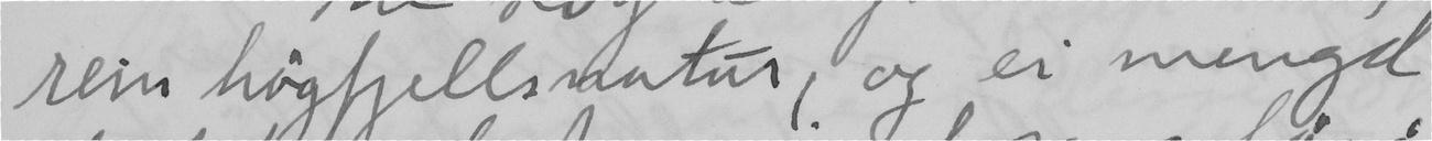rein högfjellsnatur, og ei mengd
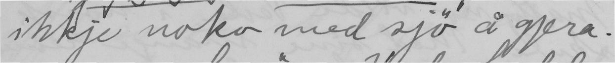ikkje noko med sjö å gjera.
